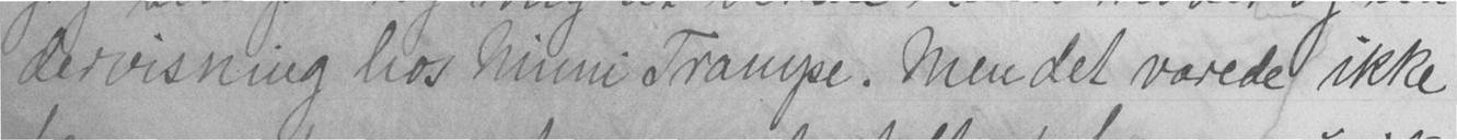dervisning hos Mimi Trampe. Men det varede ikke
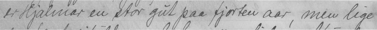er Hjalmar en stor gut paa fjorten aar, men lige
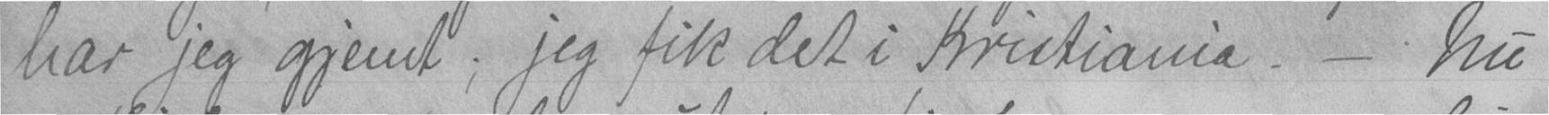har jeg gjemt; jeg fik det i Kristiania. - Nu
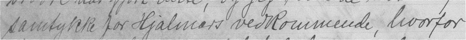samtykke for Hjalmars vedkommende, hvorfor
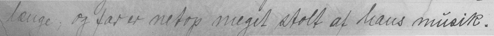længe, og far er netop meget stolt af hans musik.
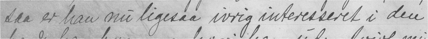saa er han nu ligesaa ivrig interesseret i den
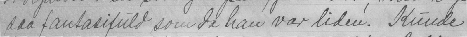saa fantasifuld som da han var liden. Kunde
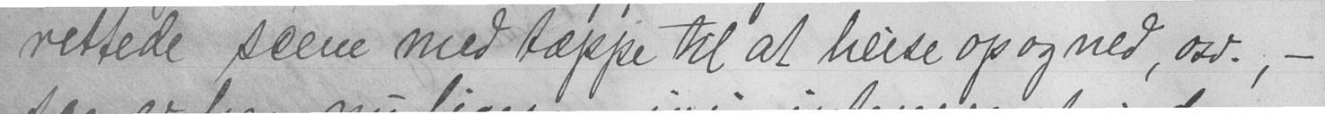rettede scene med tæppe til at heise op og ned, osv., -
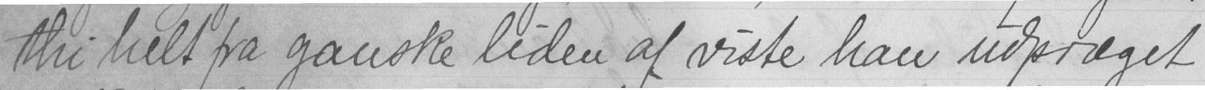thi helt fra ganske liden af viste han udpræget
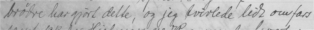brødre har gjort dette, og jeg tvivlede lidt om fars
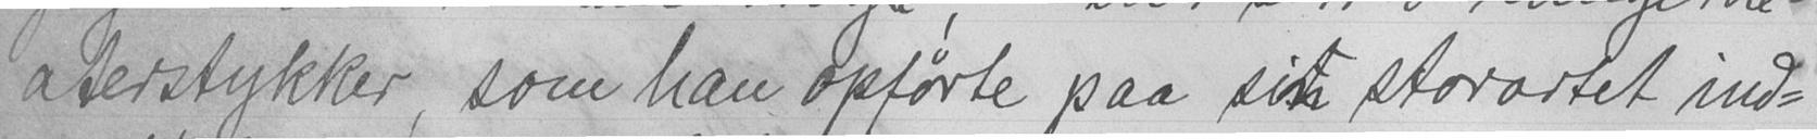aterstykker, som han opførte paa sitn storartet ind-
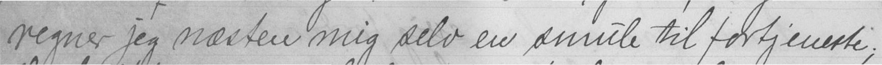regner jeg næsten mig selv en smule til fortjeneste;
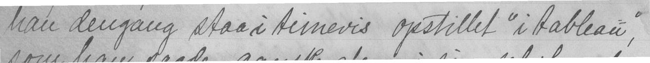han dengang staa i timevis, opstillet "i tableau,"
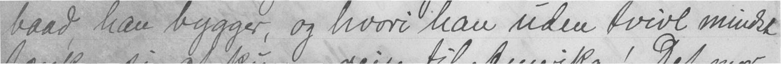baad, han bygger, og hvori han uden tvivl mindst
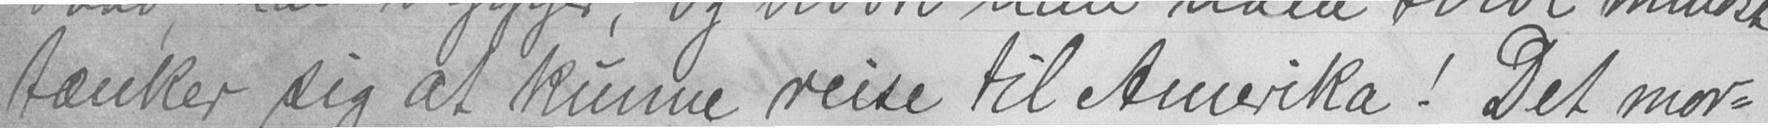tænker sig at kunne reise til Amerika! Det mor-
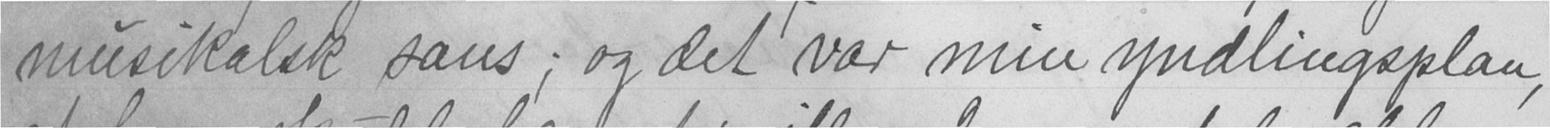musikalsk sans; og det var min yndlingsplan,
